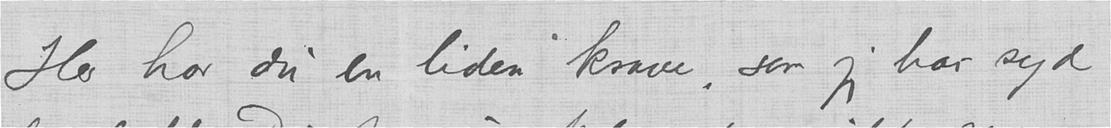Her har du en liden krave, som jeg har syd
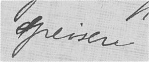peisen.
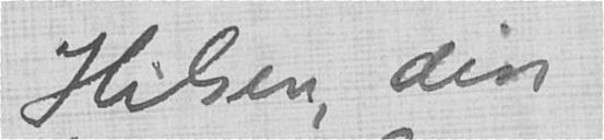Hilsen, din
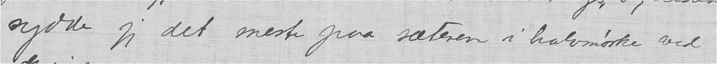sydde jeg det meste paa sæteren i halvmørke ved
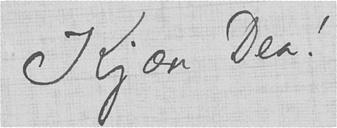Kjære Dea!
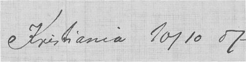Kristiania 10/10 07
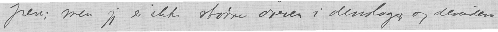pen; men jeg er ikke større dreven i denslags og desuden
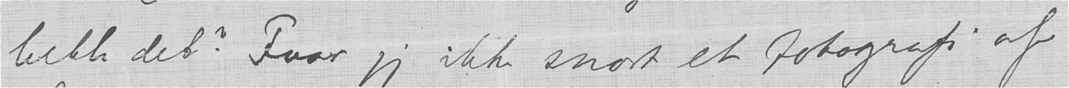beth det? Faar jeg ikke snart et fotografi af
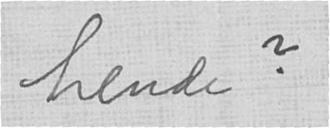hende?
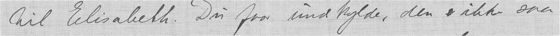til Elisabeth. Du faar undskylde, den er ikke saa
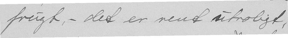frugt, - det er rent utroligt,
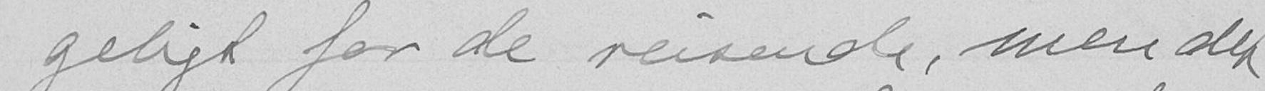geligt for de reisende, men det
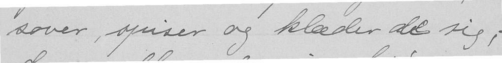sover, spiser og klæder de sig;
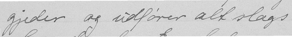gjeder og udfører alt slags
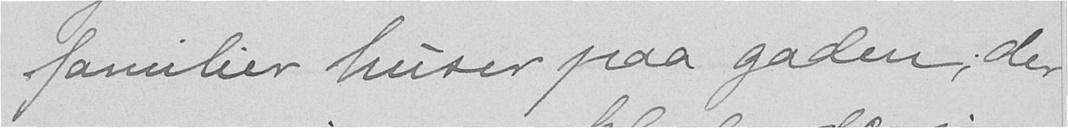familier huser paa gaden, der
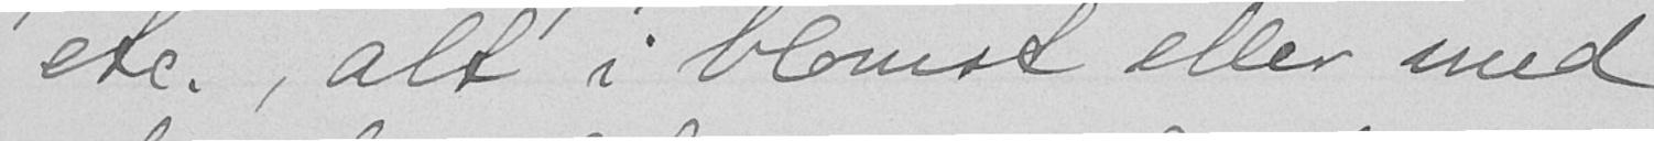etc., alt i blomst eller med
